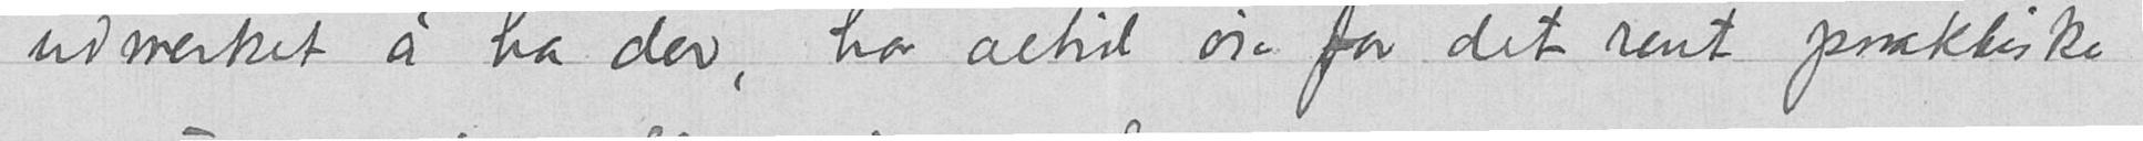udmerket å ha der, har altid øie for det rent praktiske
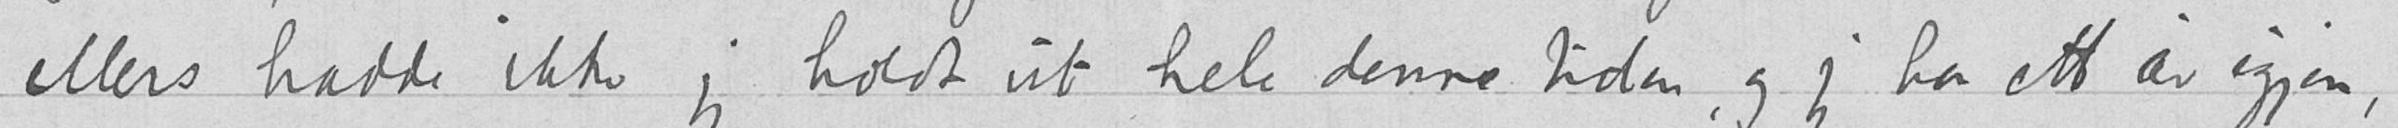ellers hadde ikke jeg holdt ut hele denne tiden, og jeg har ett år igjen,
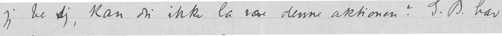jeg be dig, kan du ikke la være denne aktionen? G.B. har
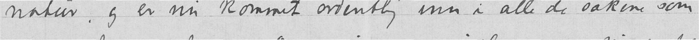natur, og er nu kommet ordentlig inn i alle de sakene som
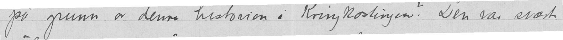få grunn av denne historien i Kringkastingen? Den var svært
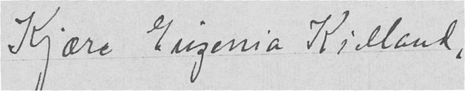Kjære Eugenia Kielland,
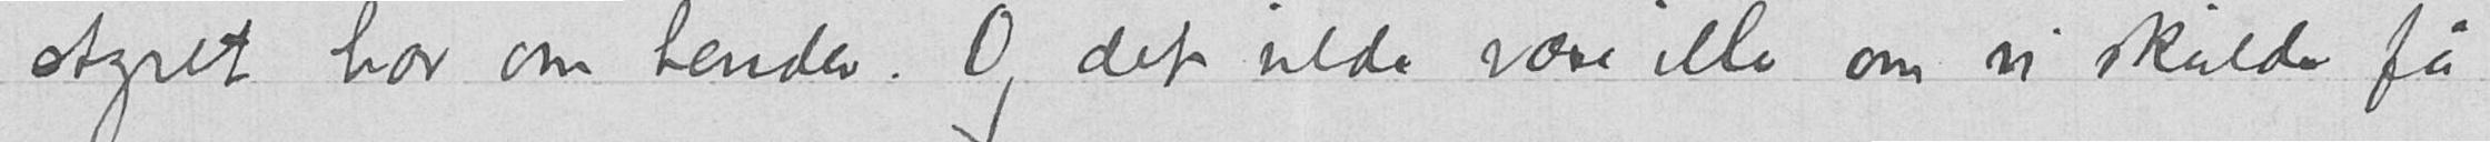styret har om hender. Og det vilde være ille om vi skulde få
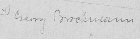Georg Brochmann
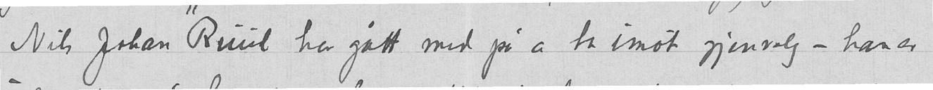Nils Johan Ruud har gått med på å ta imot gjenvalg – han er
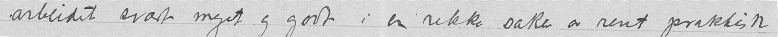arbeidet svært meget og godt i en rekke saker av rent praktisk
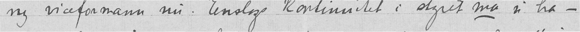ny viceformann nu. Enslags kontinuitet i styret må vi ha -
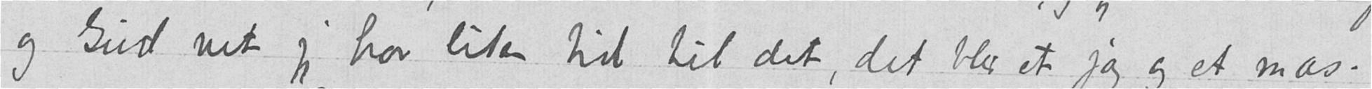og Gud vet jeg har liten tid til det, det blir et jag og et mas.
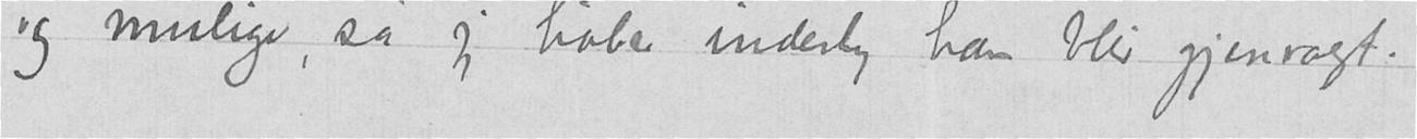og mulige, sa jeg håber inderlig han blir gjenvalgt.
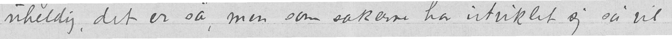uheldig, det er så, men som sakerne har utviklet sig så vil
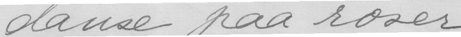danse paa roser
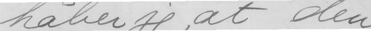håber jeg, at den
